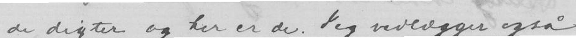de digter og her er de. Jeg vedlægger også
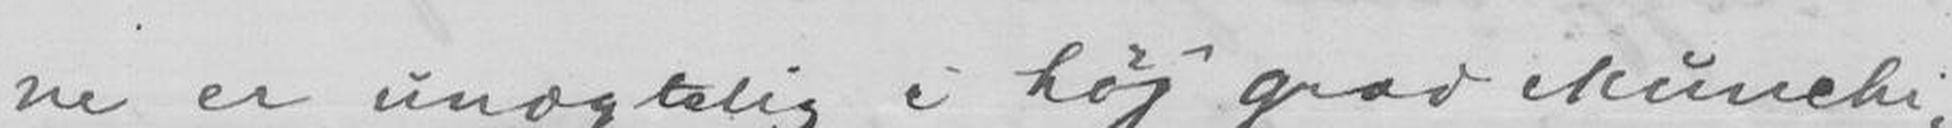ne er unægtelig i høj grad Munchi-
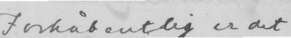Forhåbentlig er det
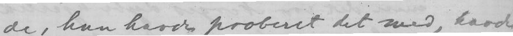de, han havde proberet det med, havde
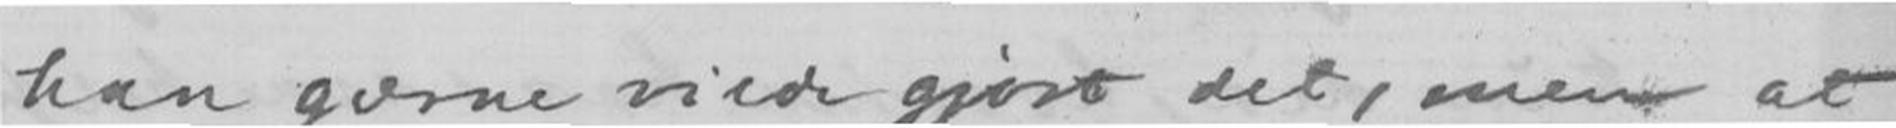han gjerne vilde gjort det, men at
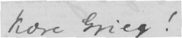Kære Grieg!
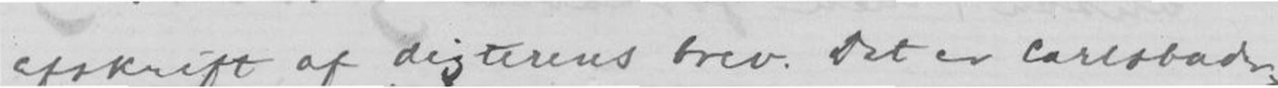afskrift af digterens brev. Det er Carlsbader-
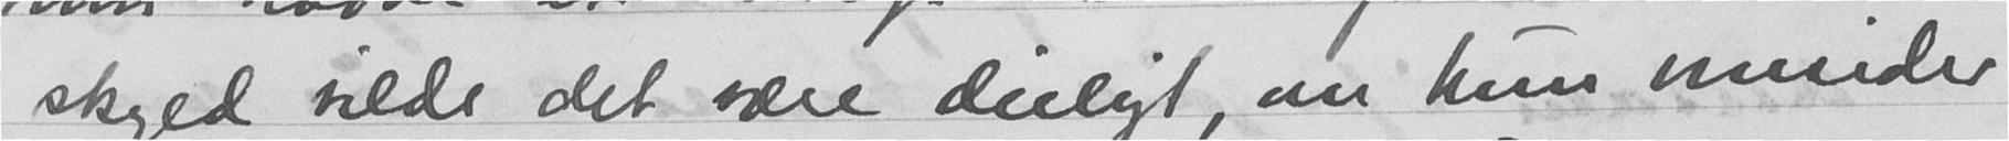skyld vilde det være deiligt, om hun omsider
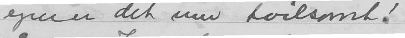egner det enn tvilsomt!
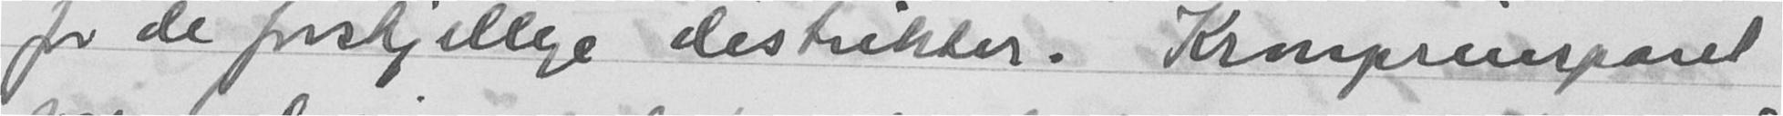for de forskjellige distrikter. Kronprinsparet
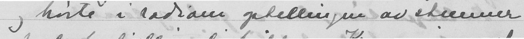og hørte i radioen optellingen av stemmer
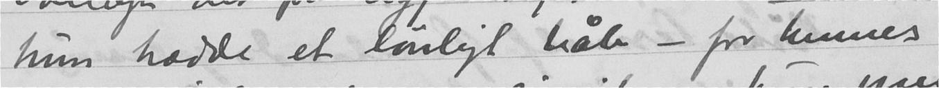hun hadde et lønligt håb - for hennes
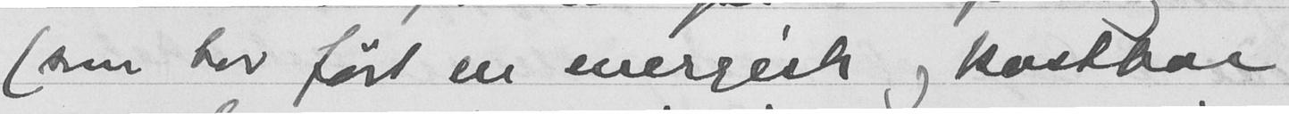(som har ført en energisk og kostbar
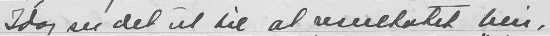Idag ser det ut til at resultatet blir,
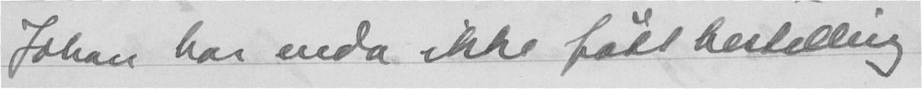Johan har enda ikke fått bestilling
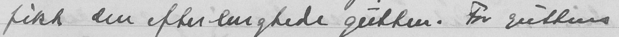fikk den efterlengtede gutten. For guttens
